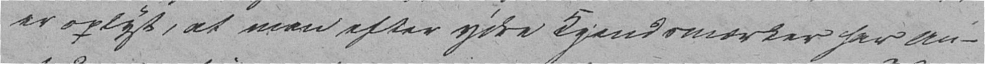er oplyst, at man efter ydre Kjendsmærker har an-
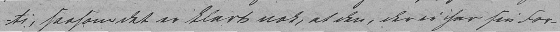ti, saasom det er klart nok, at den, der ei har sin For-
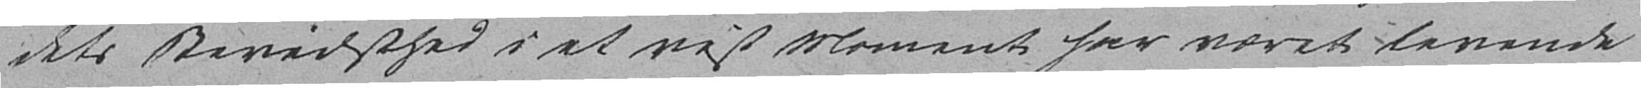dets Bevidsthed i et vist Moment har været levende
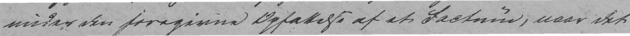under den foregivne Opfattelse af et Factum, naar det
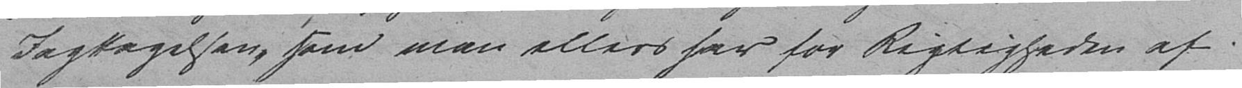Iagttagelsen, som man ellers har for Rigtigheden af
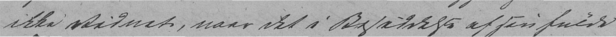ikke Vidnet, naar det i Besiddelse af sin fulde
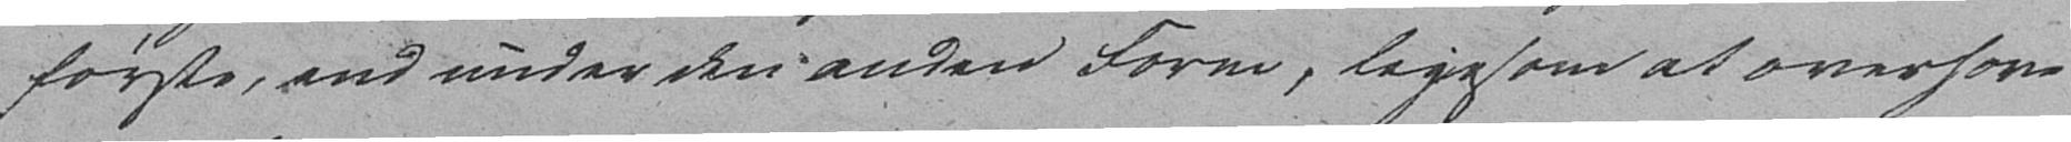første, end under den anden Form, ligesom at overhov-
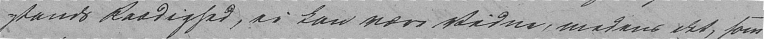stands Raadighed, ei kan være Vidne, medens det, som
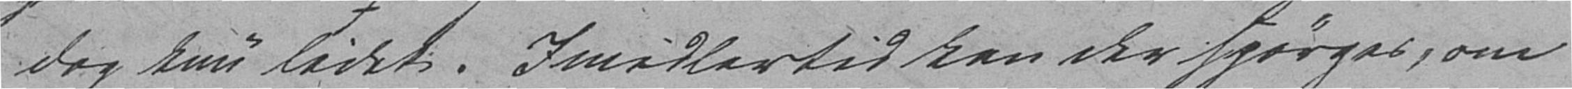dog kun lidet. Imidlertid kan der spørges, om
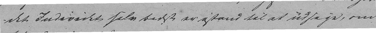edet Individet selv bedst er istand til at udsige, om
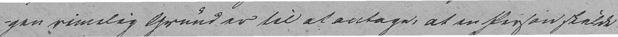gen rimelig Grund er til at antage, at en Person skulde
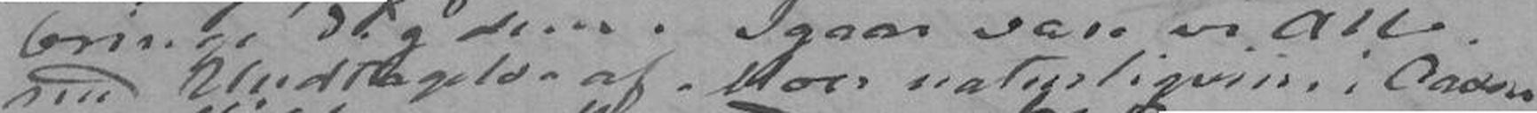med Undtagelse af Moer naturligvaas i Aasen
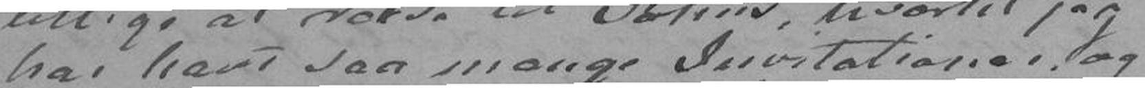har havt saa mange Invitationer, og
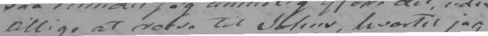tillige at reise til Johns, hvortil jeg
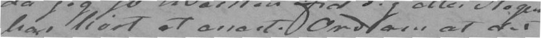har hørt et eneste Ord om at det
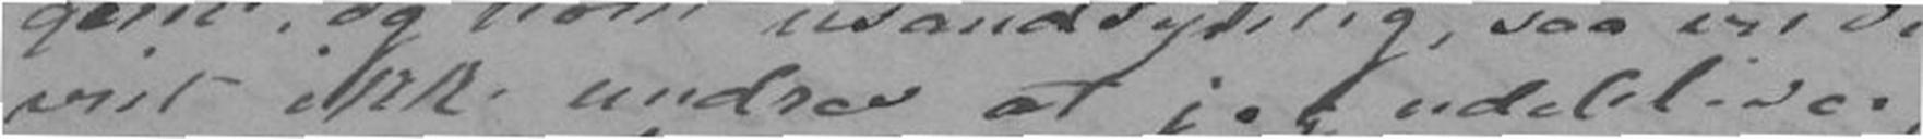vist ikke undres at jeg udebliver,
